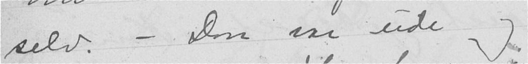selv. - Don var ude og
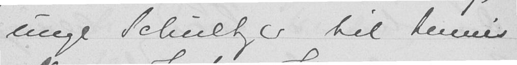unge Schultz er til tennis
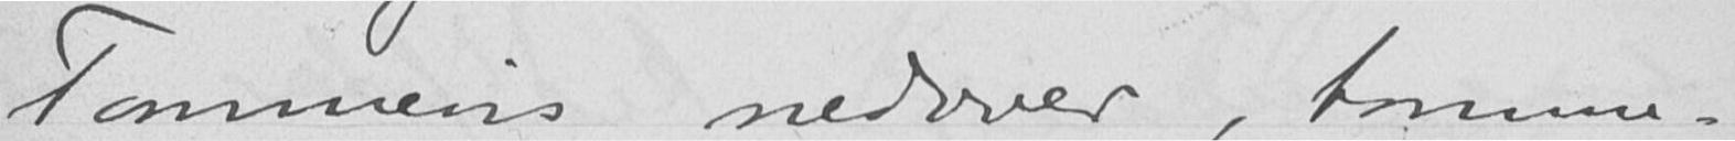Tommevis nedover, tomme-
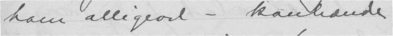ham alligevel - kanhænde
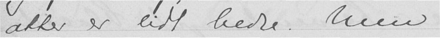atter er lidt bedre. Men
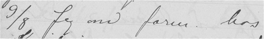9/8 Jeg om form. hos
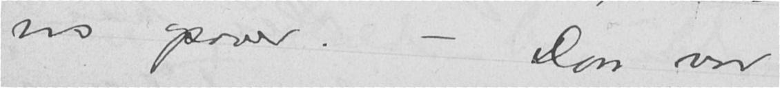vis opover. - Don var
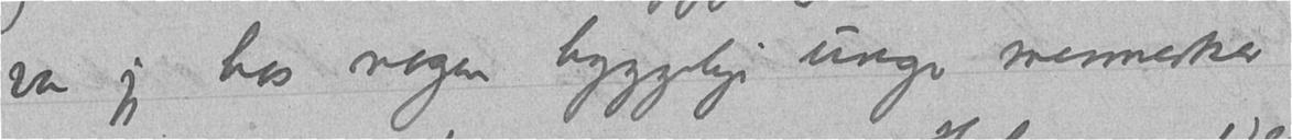var jeg hos nogen hyggelige unge mennesker
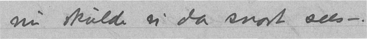nu skulde vi da snart sees –.
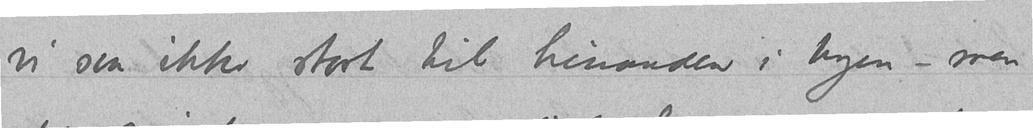vi saa ikke stort til hinanden i byen – men
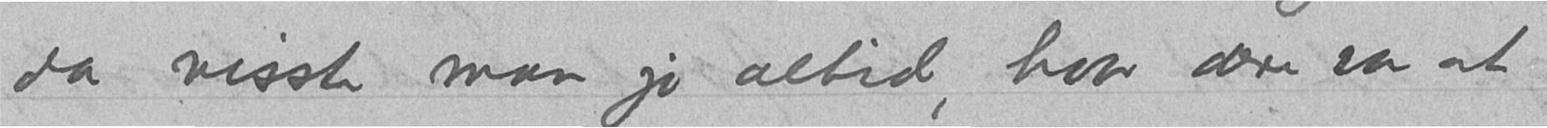da visste man jo altid, hvor dere var at
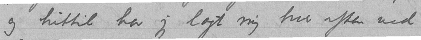og hittil har jeg lagt mig hver aften ved
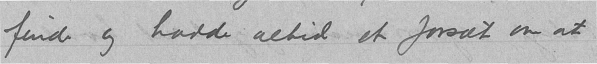finde og hadde altid et forsæt om at
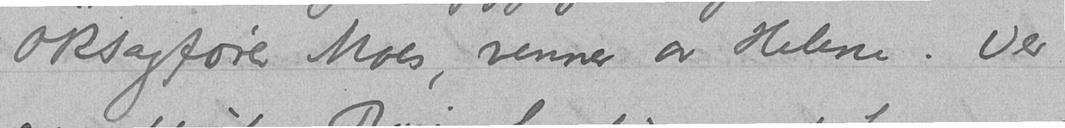ORsagfører Moes, venner av Helene. Der
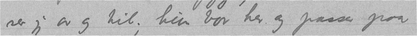ser jeg av og til, hun bor her og passer paa
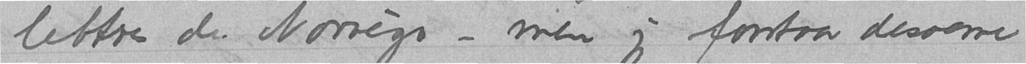lettres de Norveges – men jeg forstaar desværre
